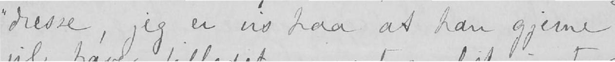dresse, jeg er vis paa at han gjerne
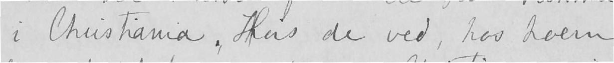i Christiania. Hvis de ved, hos hvem
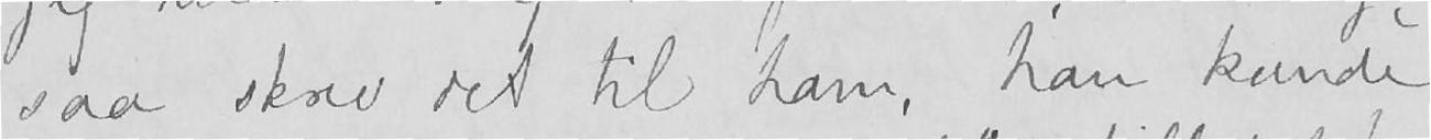saa skrev det til ham, han kunde
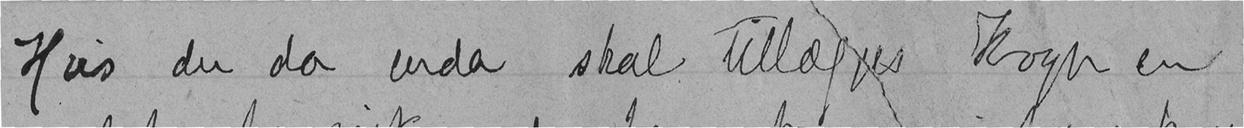Hvis du da enda skal tillægges Krogh en
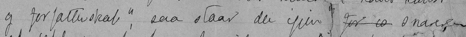og forfatterskab", saa staar der igjen" for e snarere
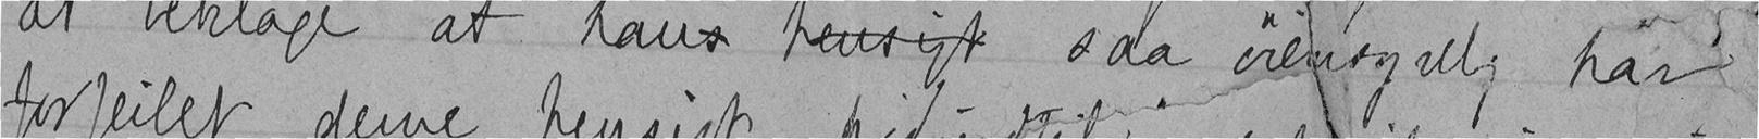at beklage, at hans hensigt saa øiensynlig har
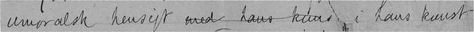umoralsk hensigt med hans kuns i hans kunst-
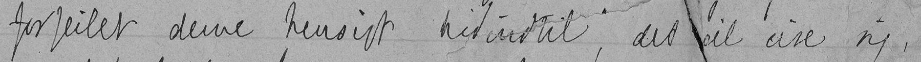forfeilet denne hensigt hidindtil, det vil vise sig,
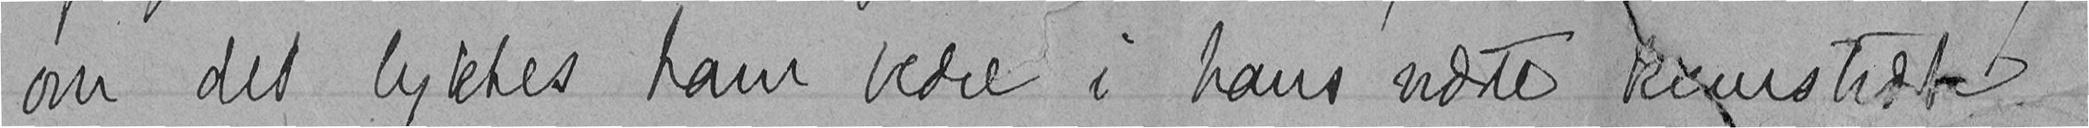om det lyktes ham bedre i hans næste kunsværk.
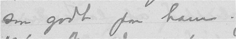saa godt paa ham.
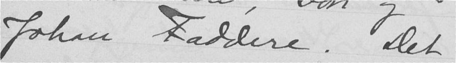Johan Faddere. Det
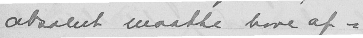absolut maatte have af-
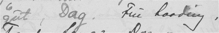gut, Dag. Fru Laading,
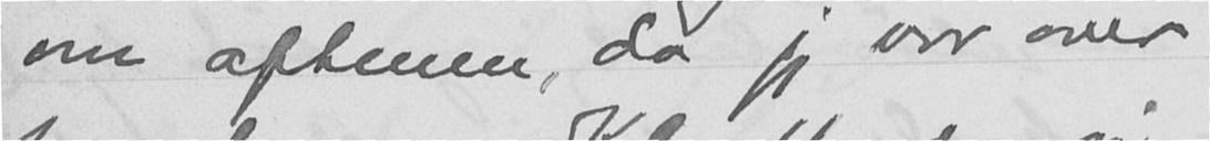om aftenen, da jeg var over
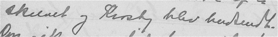skrevet og Krosby blev hendsendt.
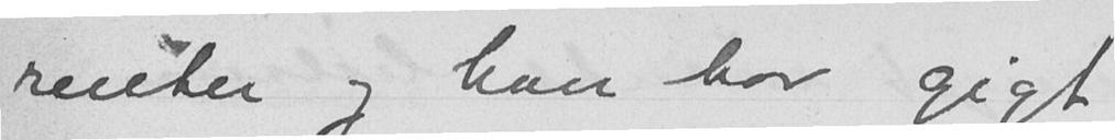renter og han har gigt
